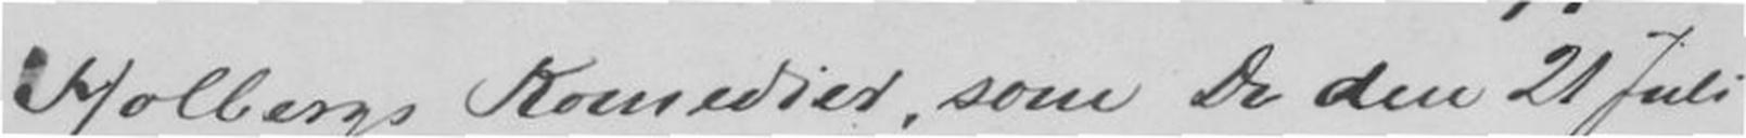Holbergs Komedier, som De den 21 Juli
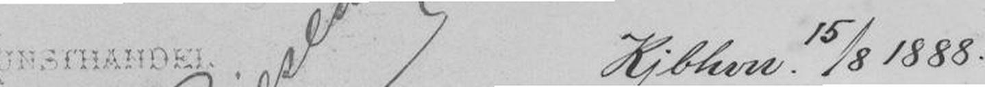KUNSTHANDELKjbhvn 15/8 1888
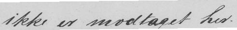ikke er modtaget her.
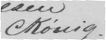König.
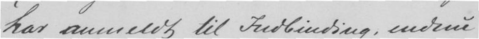har anmeldt til Indbinding, endnu
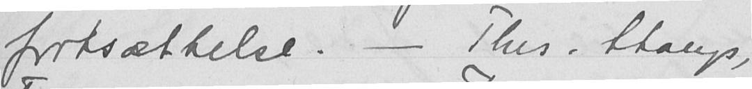fortsættelse. - Thes. Stangs
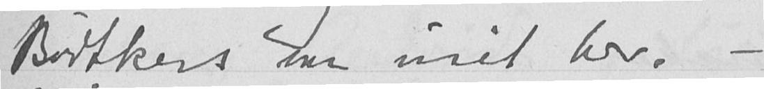Bødtkers var visit her. -
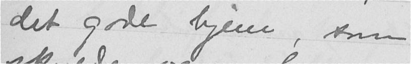det gode hjem, som
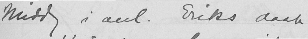Middag i anl. Eriks daab
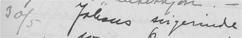30/5 Johans svigerinde
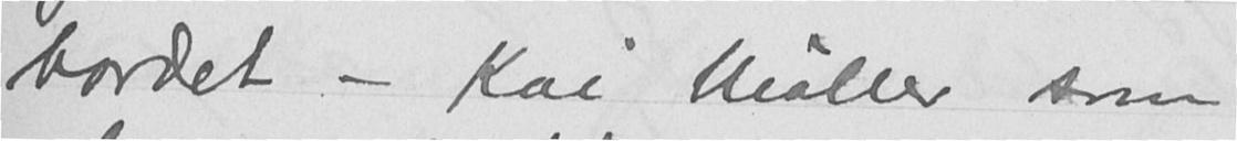bordet - Kai Møller som
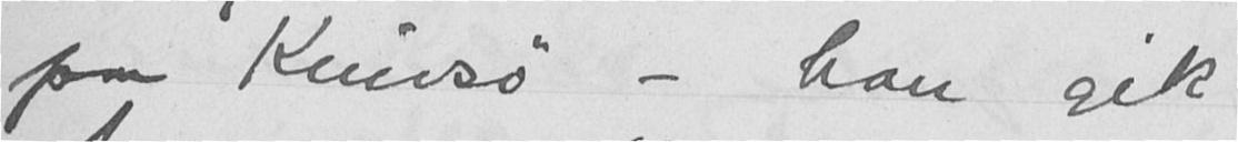paa Knivsø - han gik
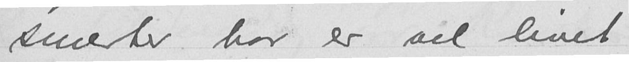smerter har er vel livet
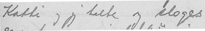Katti og jeg talte og sloges
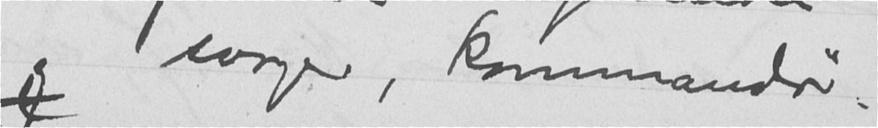og svoger, kommandør
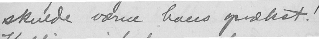skulde værne hans opvækst!
